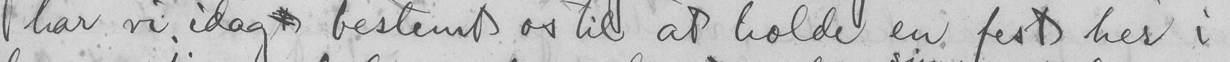har vi idag bestemt os til at holde en fest her i
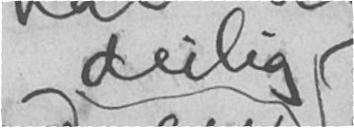deilig
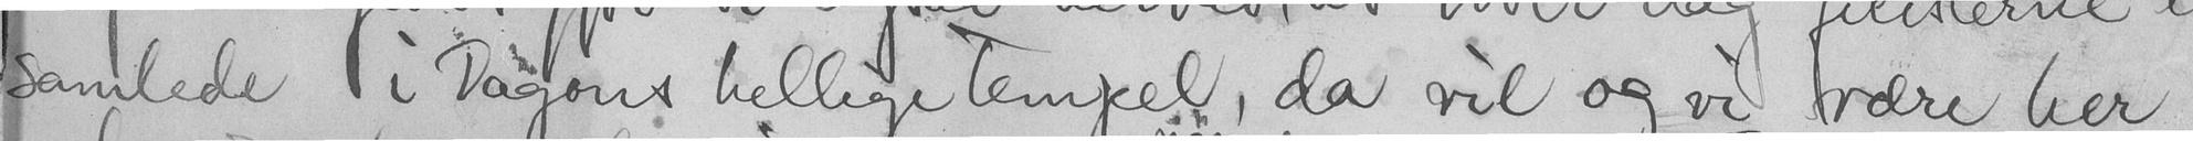samlede i Dagons hellige tempel, da vil og vi være her
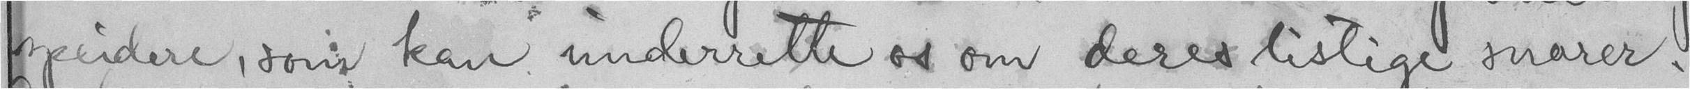speidere, som kan underrette os om deres listige snarer.
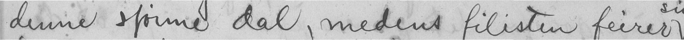denne sjønne dal, medens filisten feirer
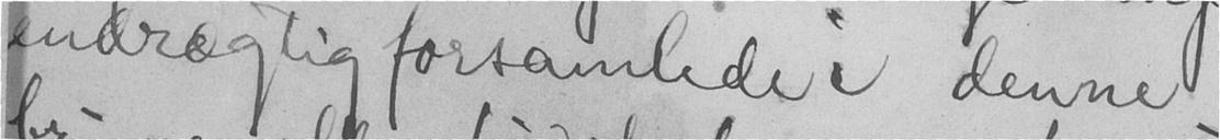endrægtig forsamlede i denne
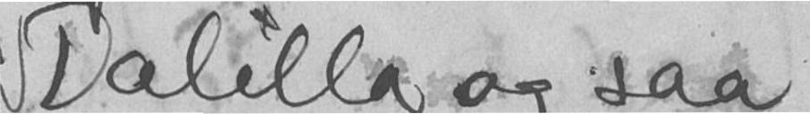Dalilla og saa
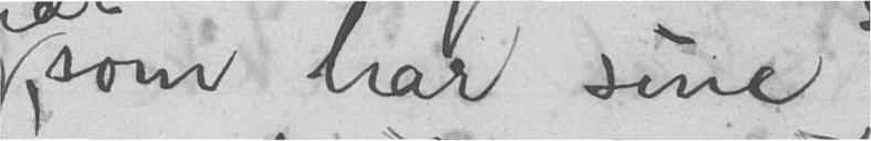som har sine
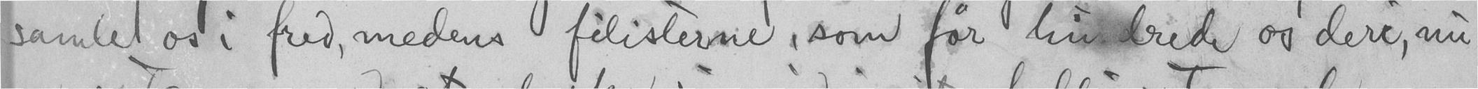samle os i fred, medens filisterne, som før hindrede os deri, nu
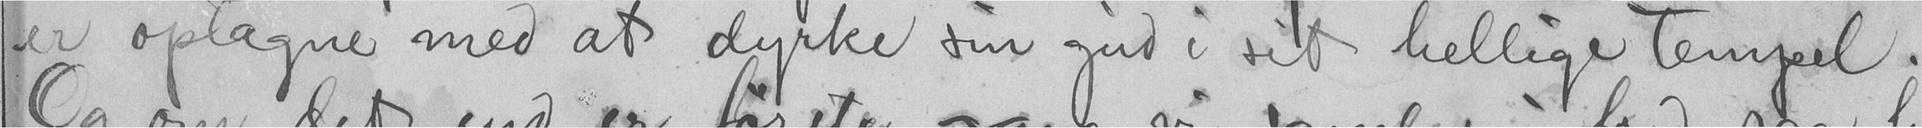er optagne med at dyrke sin gud i sit hellige tempel.
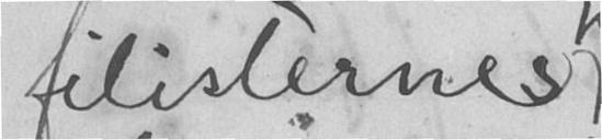filisternes
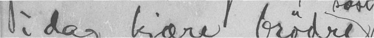i dag kjære brødre
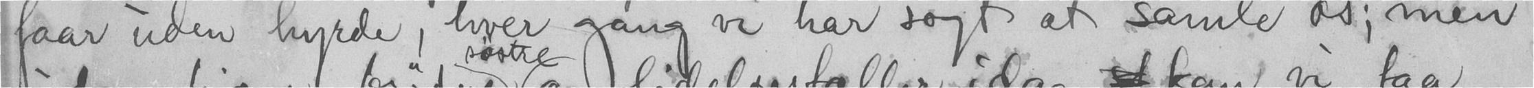faar uden hyrde, hver gang vi har søgt at samle os; men
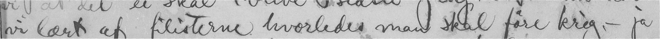vi lært af filisterne hvorledes man skal føre krig, - ja
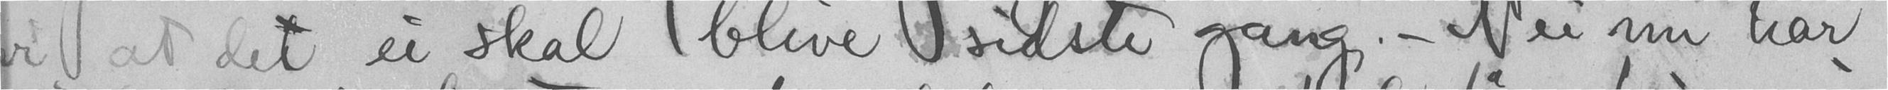vi at det ei skal blive sidste gang. - Nei nu har
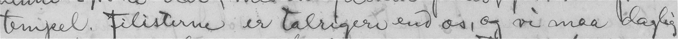tempel. Filisterne er talrigere end os, og vi maa daglig
